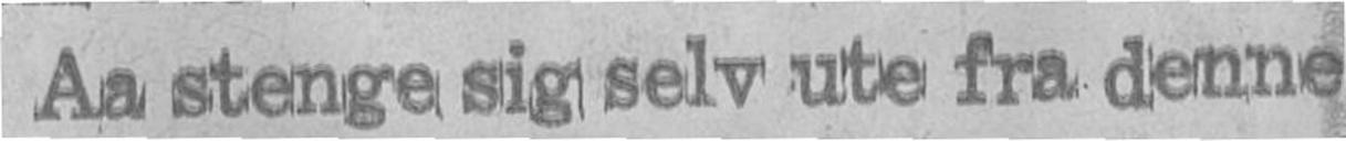Aa stenge sig selv ute fra denne
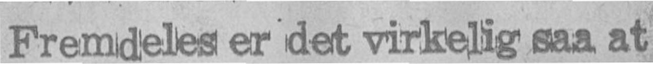Fremdeles er det virkelig saa at
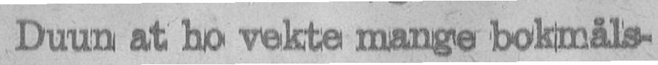Duun at ho vekte mange bokmåls-
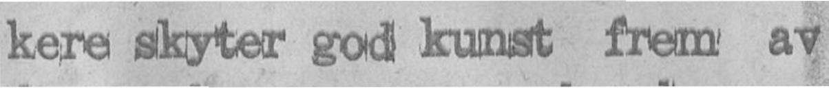kere skyter god kunst frem av
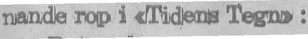nande rop i «Tidens Tegn» :
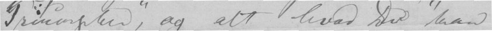Triumpher, og alt hvad Du kan
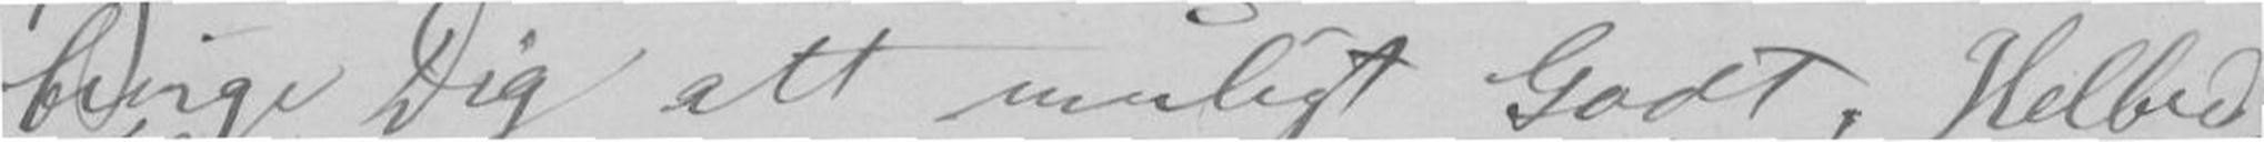bringe Dig alt muligt Godt, Helbred,
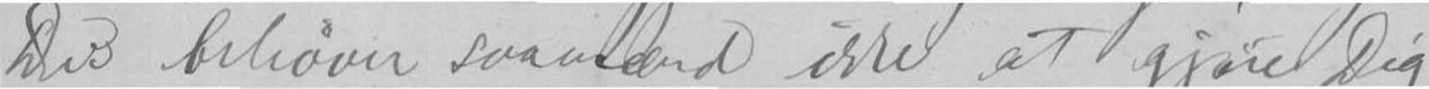Du behøver saamænd ikke at gjøre Dig
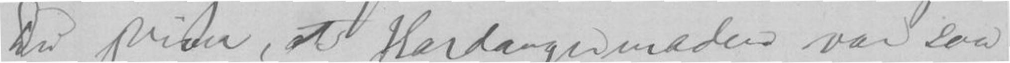Du skriver, at Hardangermaden var saa
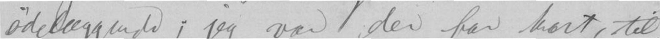ødelæggende; jeg var der for kort, til,
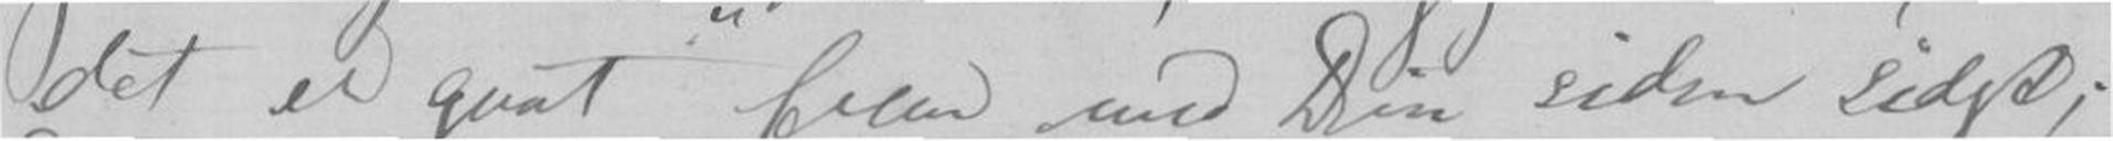det er gaat frem med Din siden sidst;
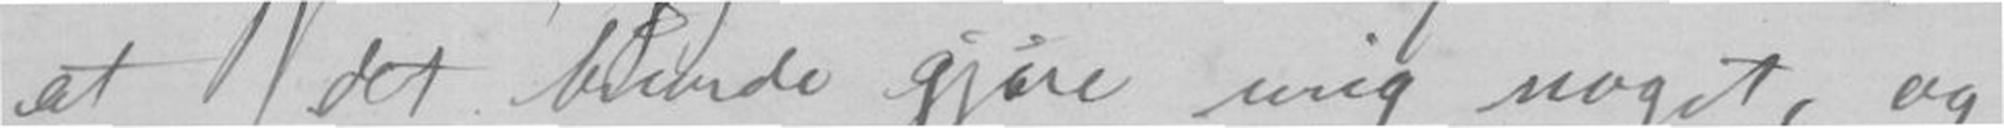at det kunde gjøre mig noget, og
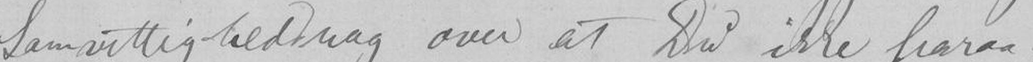Samvittighedsnag over at Du ikke fraraa
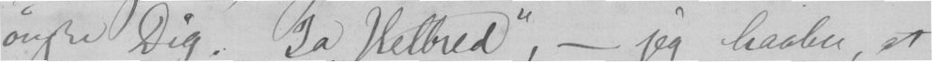ønske Dig. Ja Helbred, - jeg haaber, at
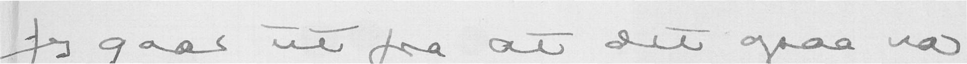Jeg gaar ut fra at du ogsaa var
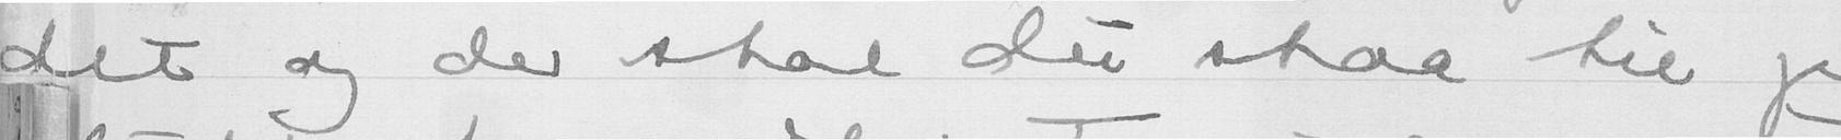det og der skal du staa til jeg
